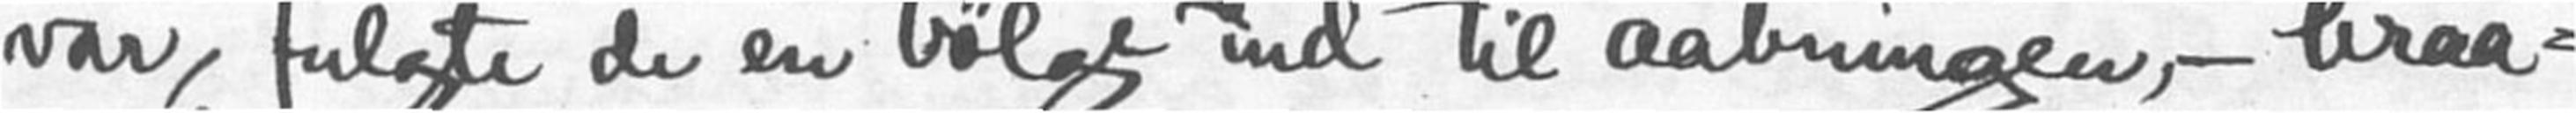var, fulgte de en bølge ind til aabningen,- braa-
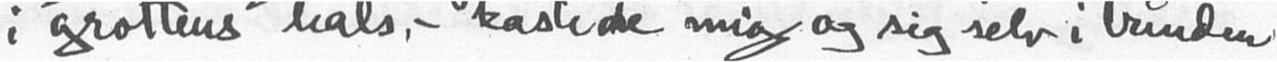i grottens hals, - kastede mig og sig selv i bunden
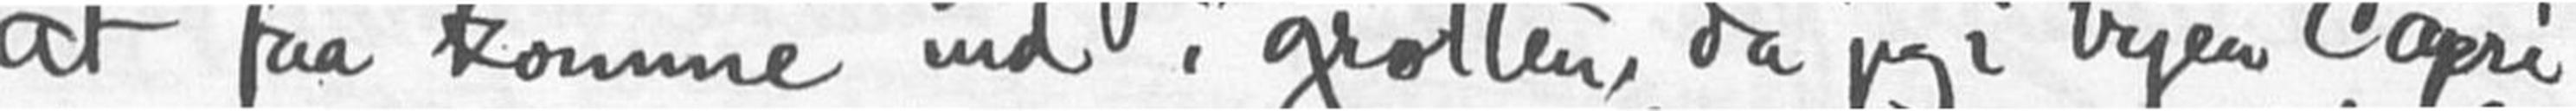at faa komme ind i grotten, da jeg i byen Capri
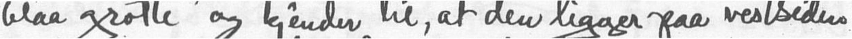"blaa grotte" og kjender til, at den ligger paa vestsiden
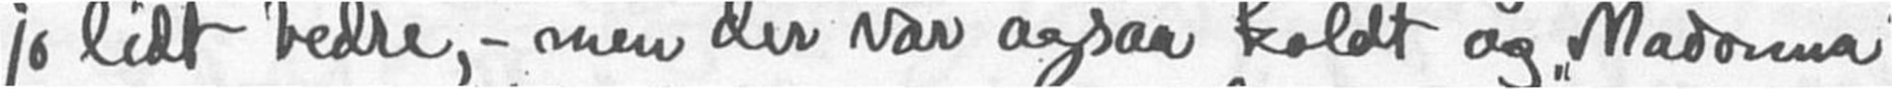jo lidt bedre, - men der var ogsaa koldt og "Madonna"
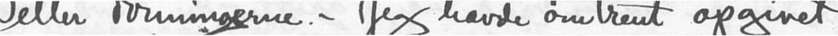eller dønningerne. - Jeg havde omtrent opgivet
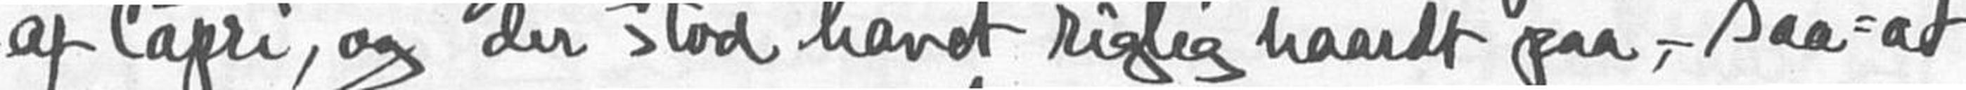af Capri, og der stod havet rigtig haardt paa, - saa at
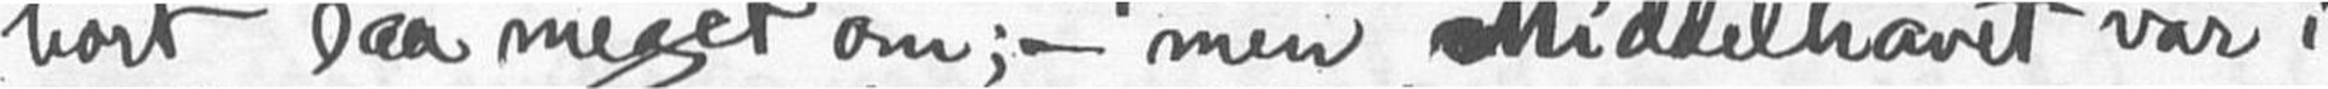hørt saa meget om, - men Middelhavet var i
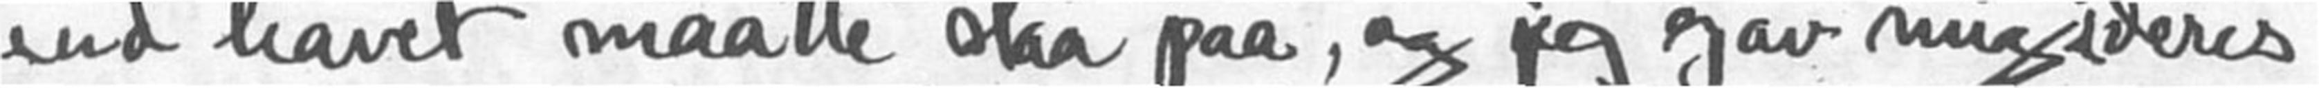end havet maatte staa paa, og jeg gav mig i deres
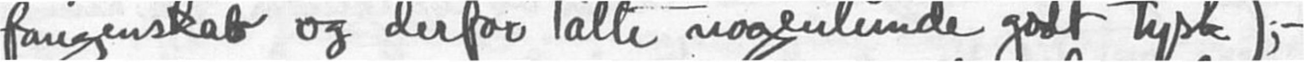fangenskab og derfor talte nogenlunde godt tysk); -
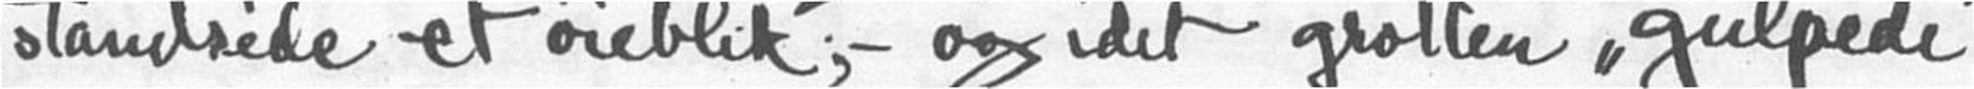standsede et øieblik, - og idet grotten "gulpede"
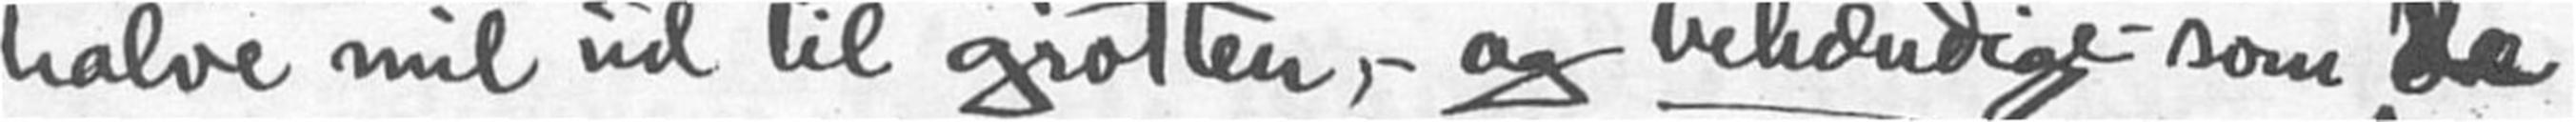halve mil ud til grotten, - og behændige som de
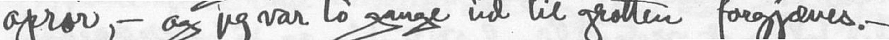oprør, - og jeg var to gange ud til grotten forgjæves.
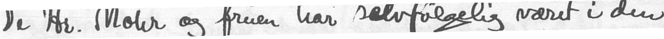De Hr. Mohr og fruen har selvfølgelig været i den
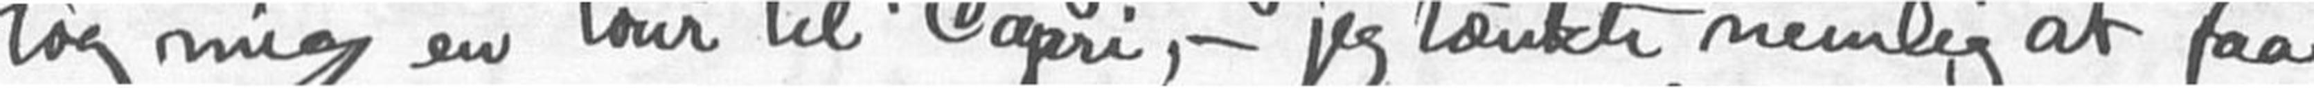tog mig en tour til Capri; - jeg tænkte nemlig at faa
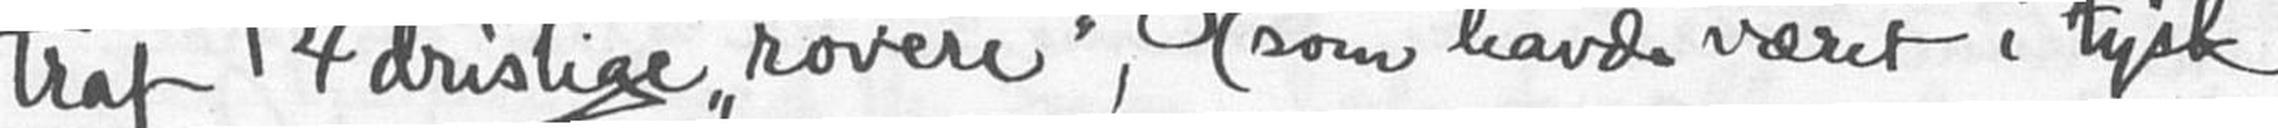traf 4 dristige "røvere", (som havde været i tysk
